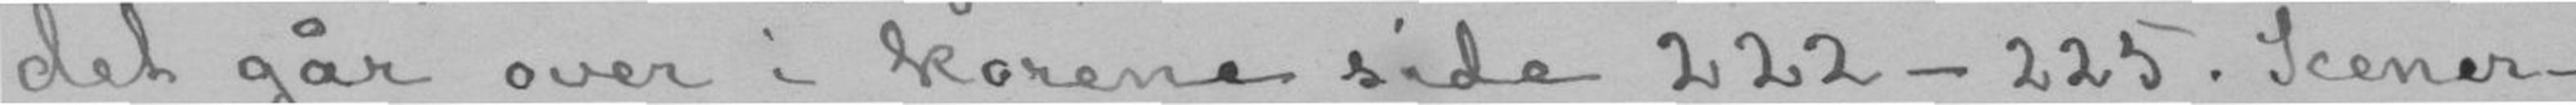det går over i korene side 222-225. Scener-
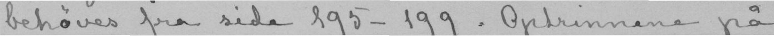behøves fra side 195-199. Optrinnene på
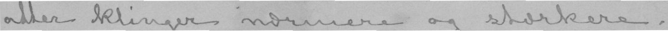atter klinger nærmere og stærkere.
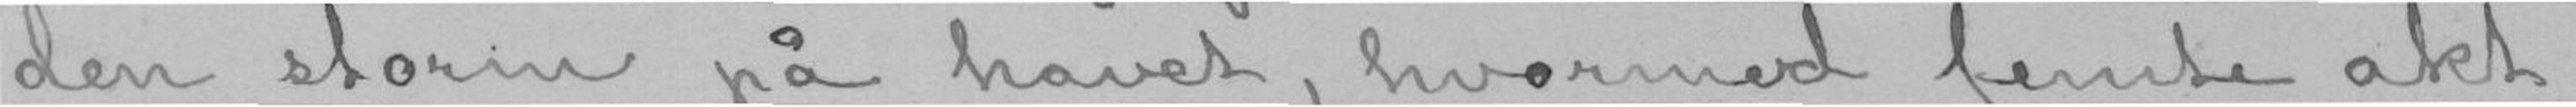den storm på havet, hvormed femte akt
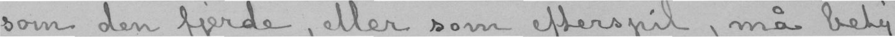som den fjerde, eller som efterspil, må bety-
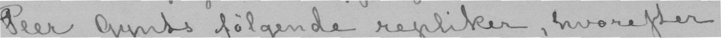Peer Gynts følgende repliker, hvorefter
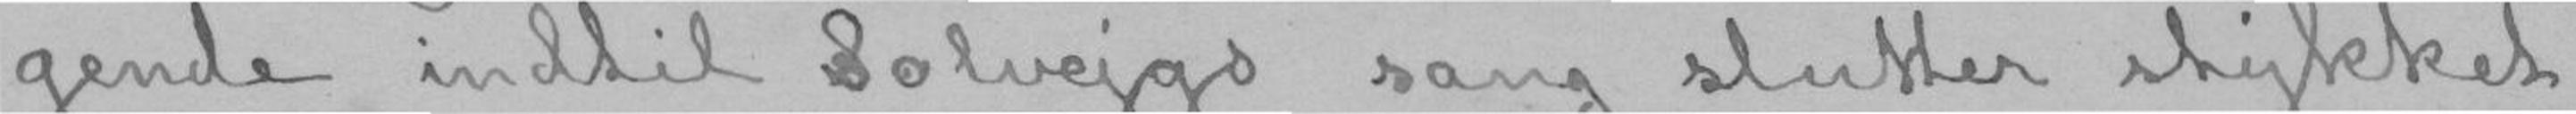gende indtil Solvejgs sang slutter stykket
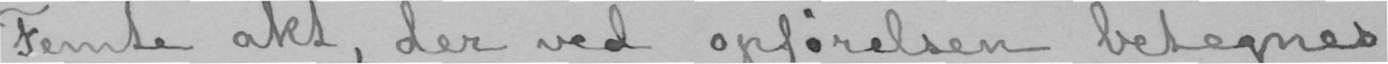Femte akt, der ved opførelsen betegnes
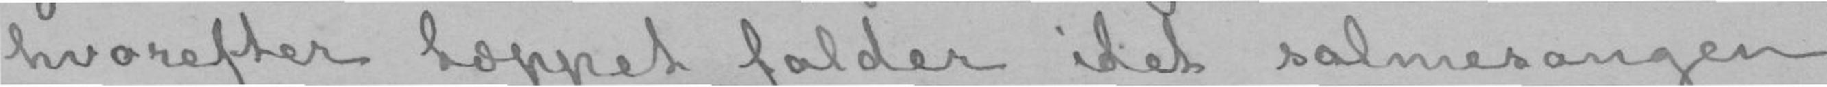hvorefter tæppet falder idet salmesangen
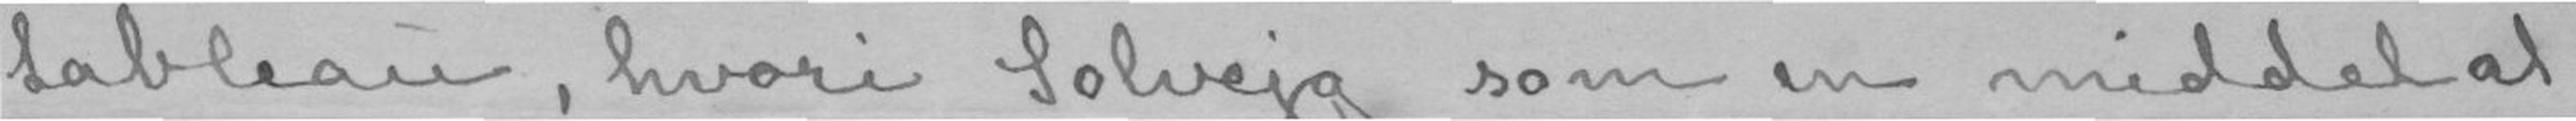tableau, hvori Solvejg som en middelal-
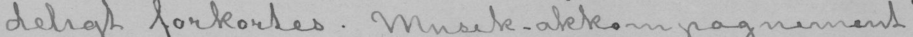deligt forkortes. Musik-akkompagnement
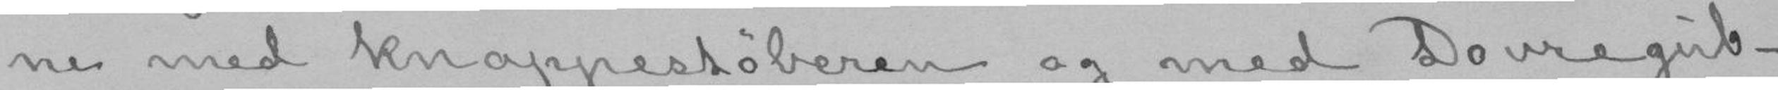ne med knappestøberen og med Dovregub-
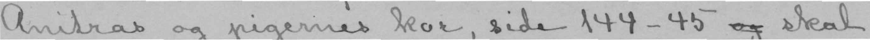Anitras og pigernes kor, side 144-45 og skal
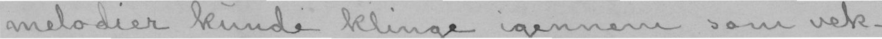melodier kunde klinge igennem som vek-
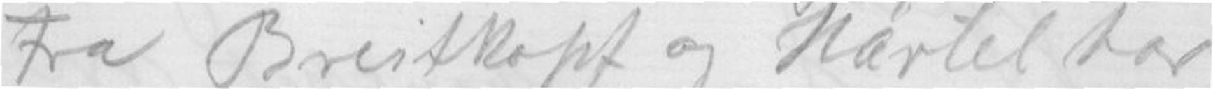Fra Breitkopf og Härtel
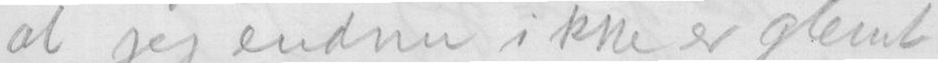at jeg endnu ikke er glemt
